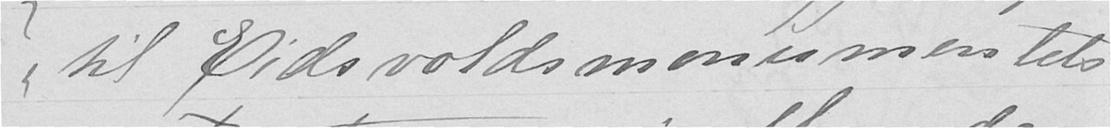til Eidsvoldsmonumentets
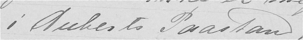i Auberts Paastand,
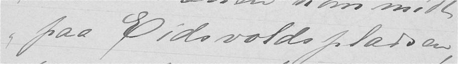paa Eidsvoldspladsen,
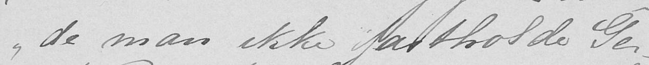de man ikke fastholde Ger-
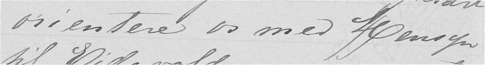orientere os med Hensyn
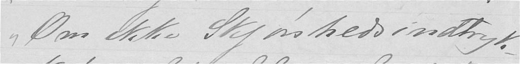Om ikke Skjønhedsindtryk-
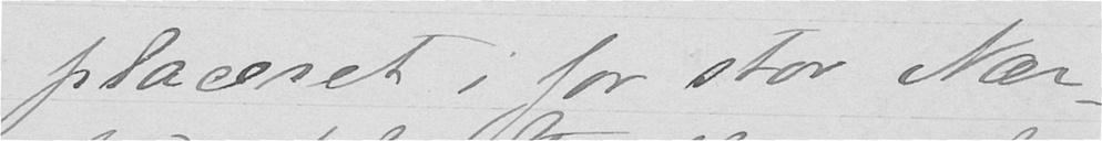placeret i for stor Nær-
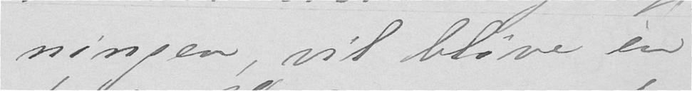ningen, vil blive en
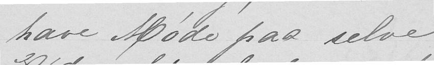have Møde paa selve
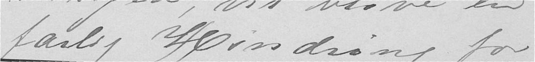farlig Hindring for
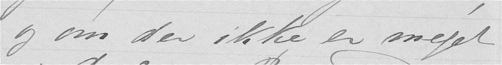og om der ikke er meget
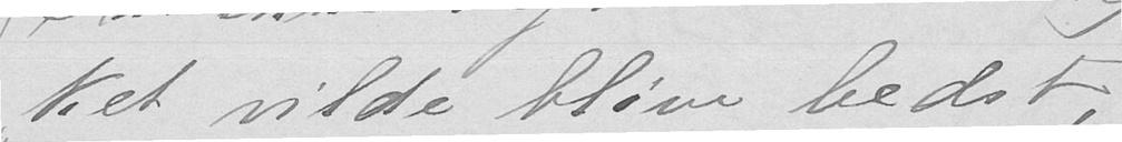ket vilde blive bedst,
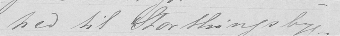hed til Storthingsbyg-
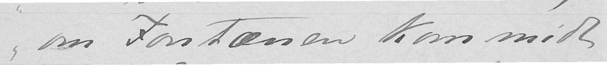om Fontænen kom midt
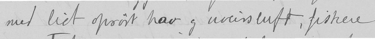med lidt oprørt hav og uveirsluft, fiskere
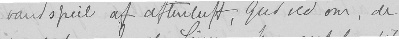vandspeil af aftenluft, Gud ved om, de
